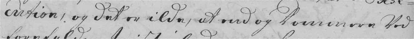cution, og det er ilde, at end og Dommere Ved
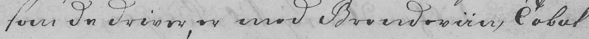som de driver, er med Brendeviin, Tobak
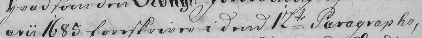arii 1685 foreskriver i dend 12te Paragrapho,
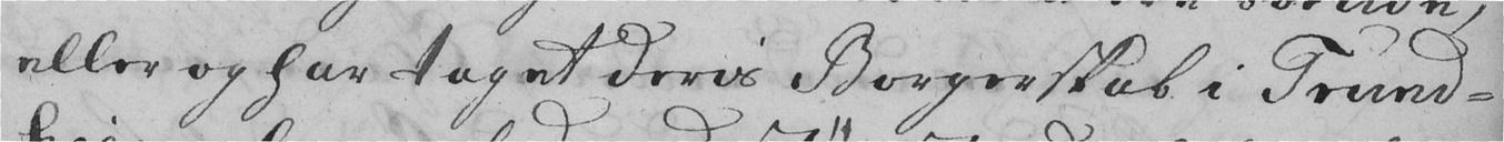eller og har taget deris Borgerskab i Trund-
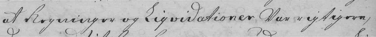at Regninger og Liqvidationer Var rigtigere,
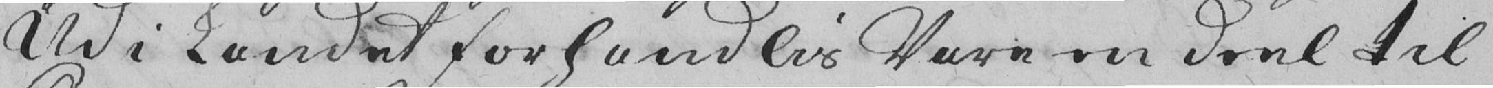Ud i Landet forhandlis Vare en deel til
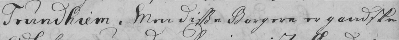Trundhiem. Men disse Borgere er gandske
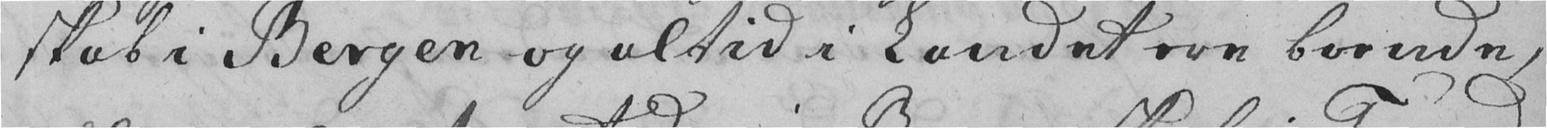skab i Bergen og altid i Landet ere boende,
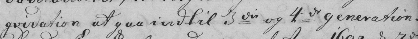qvidation at gaa indtil 3die og 4de generation.
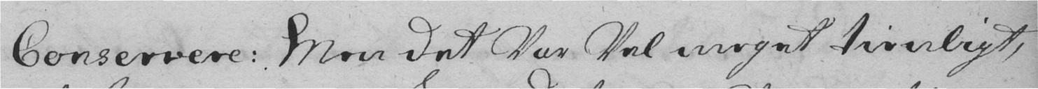Conservere: Men det Var Vel meget tienligt,
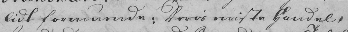lidt formuende. Deris eniste Handel,
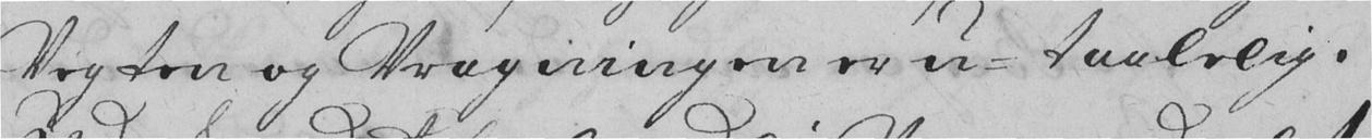Vegten og Vragningen er u-taalelig.
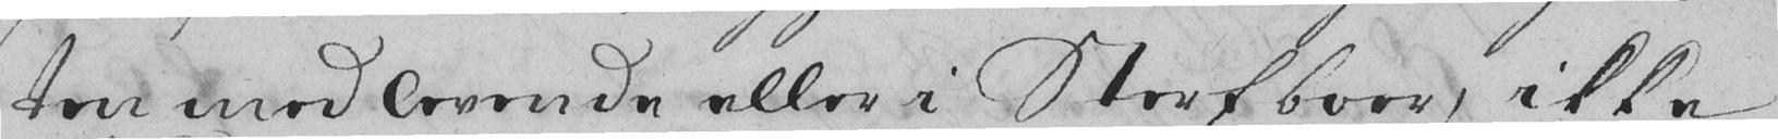ten med levende eller i Stort boar, ikke
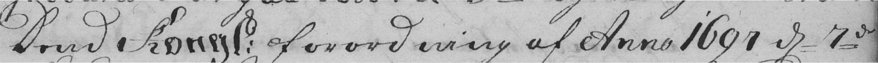Dend Kongl. Forordning af Anno 1697 d 7de
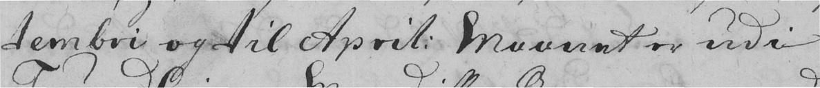tembri og til April Maanet er udi
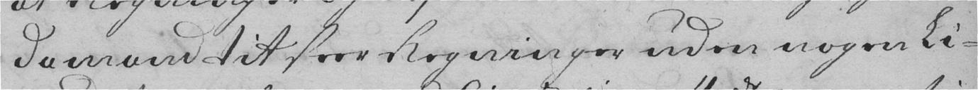da mand tit seer Regninger uden nogen Li-
